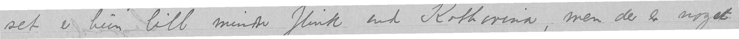set er hun litt mindre flink end Katharina, men der er noget
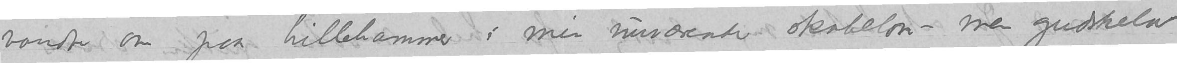vandre om paa Lillehammer i min nuværende skabelon – men gudskelov
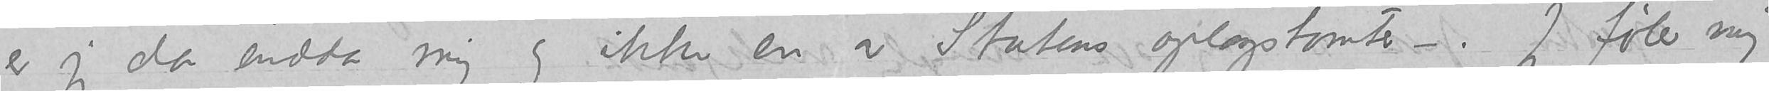er jeg da endda mig og ikke en av Statens oplagstomter –. Jeg føler mig
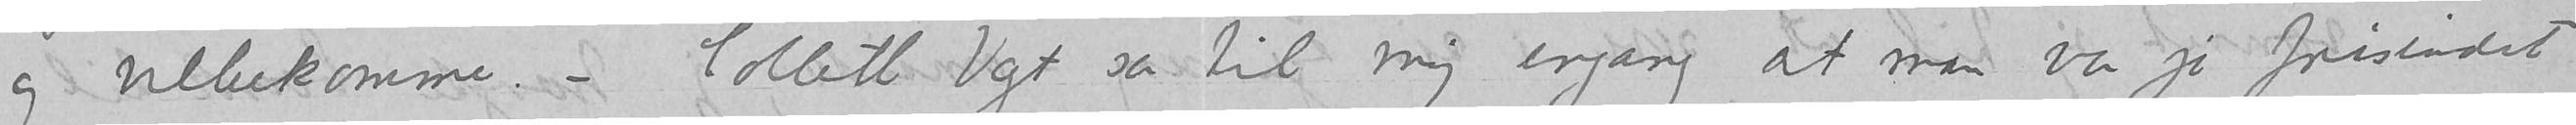og velbekomme. – Collett Vogt sa til mig engang at man var jo frisindet
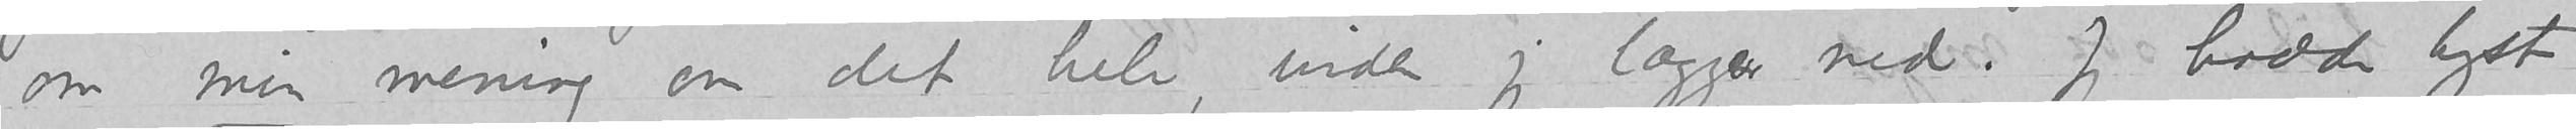om min mening om det hele, inden jeg lægger ned. Jeg hadde lyst
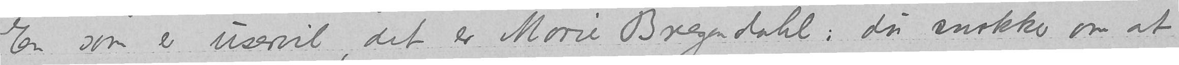En som er uservil, det er Marie Bregendahl: du snakker om at
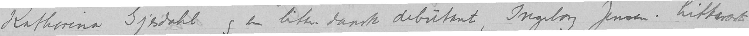Katharina Gjesdahl og en liten dansk debutant, Ingeborg Jensen. Litterært
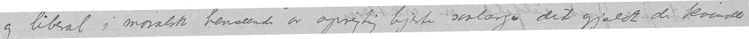og liberal i moralsk henseende av oprigtig hjerte saalænge det gjaldt de kvinder
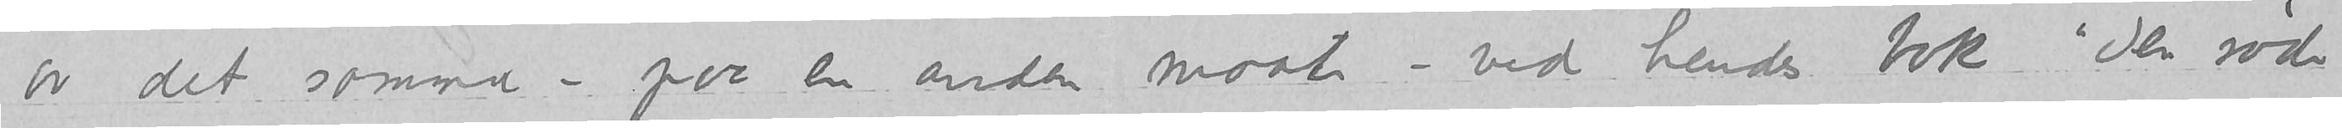av det samme – paa en anden maate – ved hendes bok «Den røde
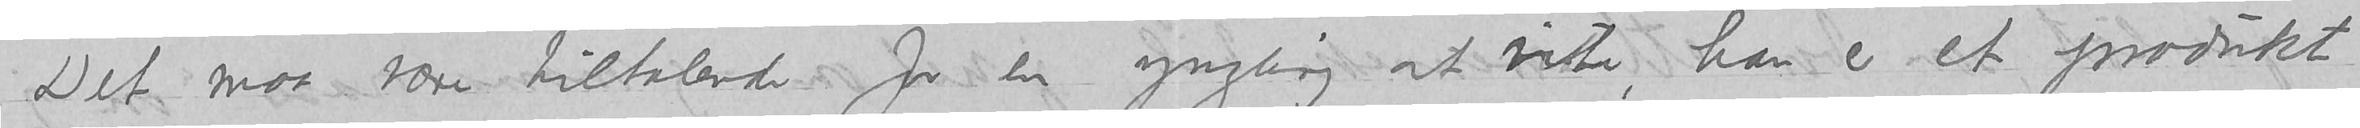Det maa være tiltalende for en yngling at vite, han er et produkt
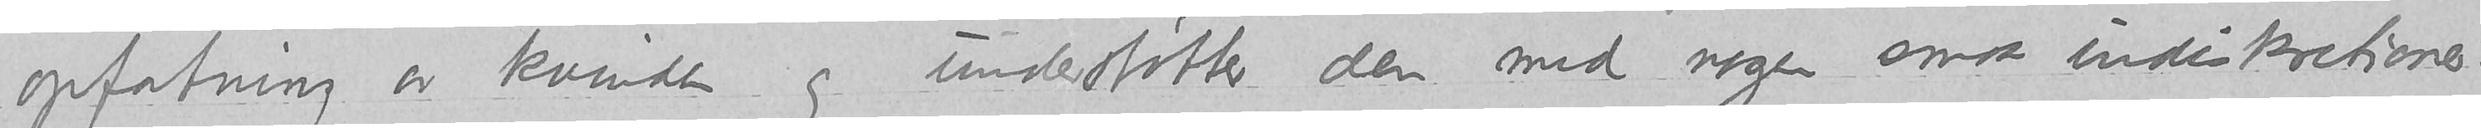opfatning av kvinden og understøtter den med nogen smaa indiskretioner.
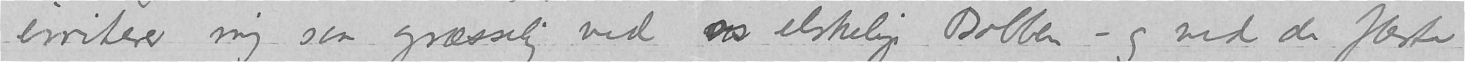irriterer mig saa græsselig ved saa elskelige Dolben – og ved de fleste
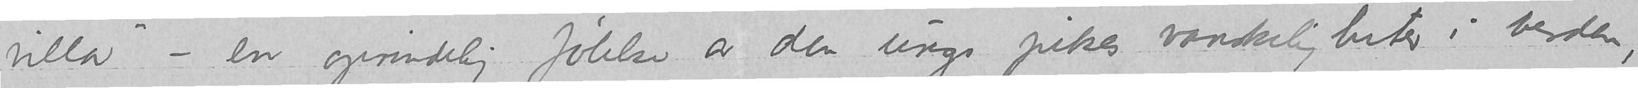villa» – en oprindelig følelse av den unge pikes vanskeligheter i verden,
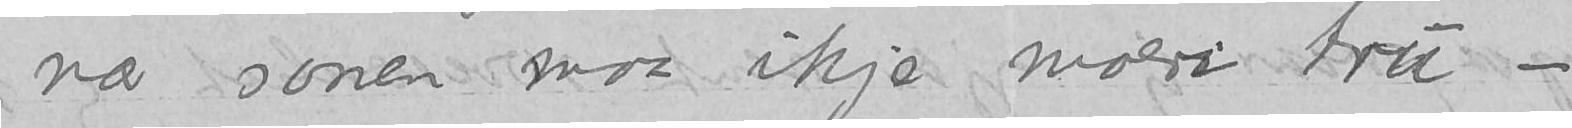nær sonen maa ikje moeri tru –
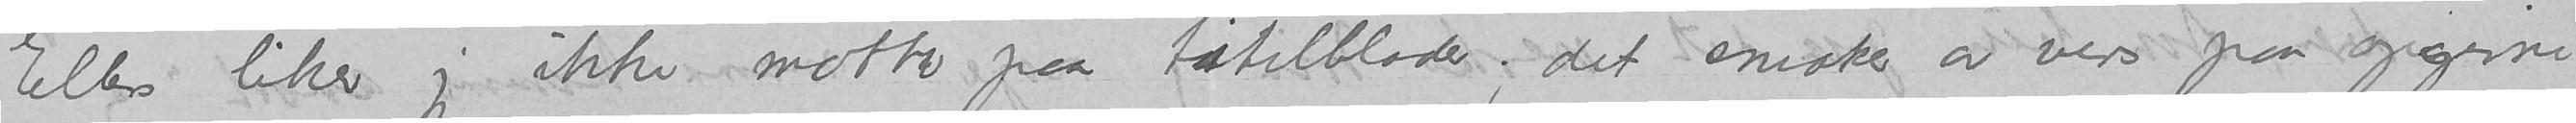Ellers liker jeg ikke motto paa titelblader; det smaker av vers paa opgivne
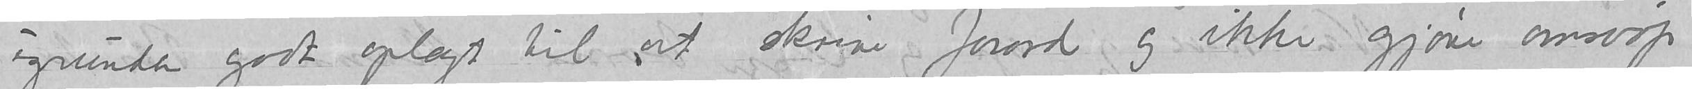igrunden godt oplagt til at skrive forord og ikke gjøre omsvøp
